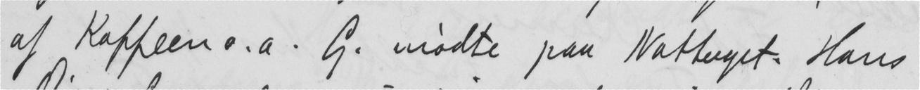af Kaffeen o.a. G. mødte paa Nattoget. Hans
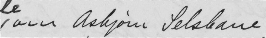om Asbjørn Selsbane
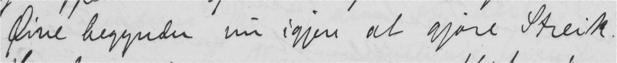Øine begynder nu igjen at gjøre Streik.
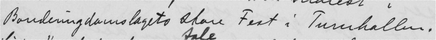Bondeungdomslagets store Fest i Turnhallen.
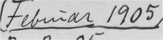Februar 1905
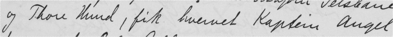og Thore Hund, fik hvervet Kaptene Angel
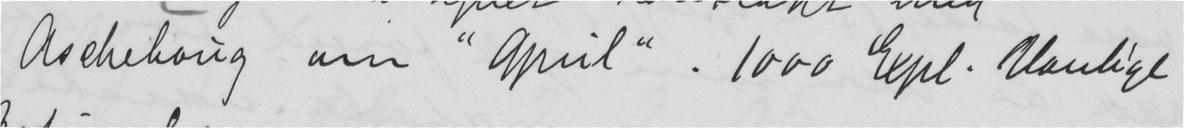Aschehoug om "April". 1000 Expl. Vanlige
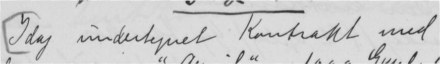Idag undertegnet Kontrakt med
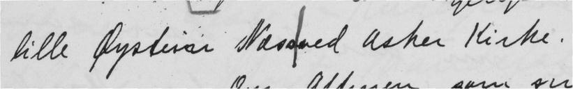lille Øystein Næss ved Asker Kirke.
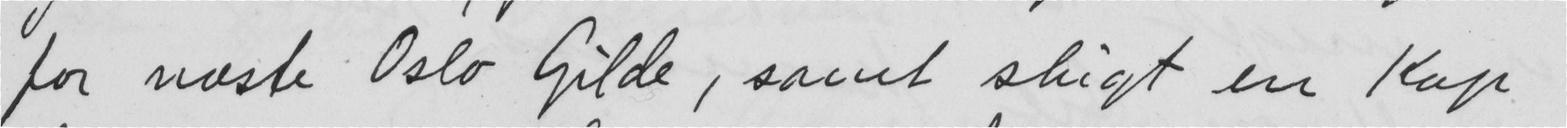for næste Oslo Gilde, samt slugt en Kop
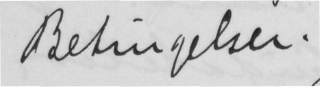Betingelser!
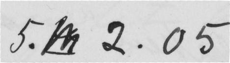5. 2.05
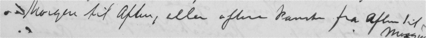Morgen til Aften, eller oftere kanske fra Aften til
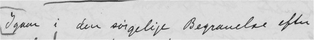Igaar i den sørgelige Begravelse efter
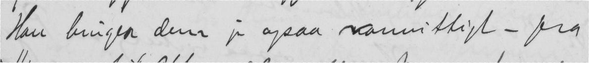Han bruger dem jo ogsaa vanvittigt - fra
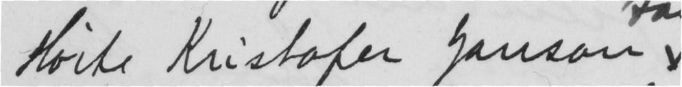Hørte Kristofer Janson
